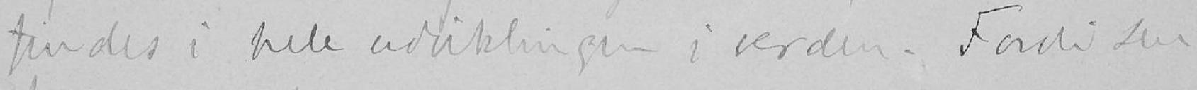findes i hele udviklinger i verden. Fordi Du
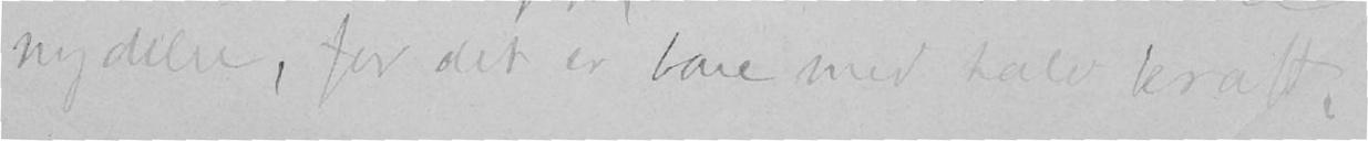nydelse, for det er bare med halv kraft,
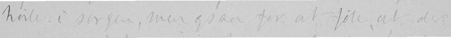hvile i sorgen, men ogsaa for at føle, at der
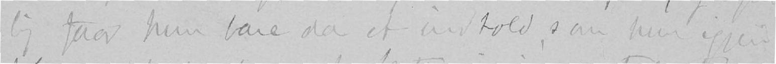lig faar hun bare da et indhold, som hun igjen
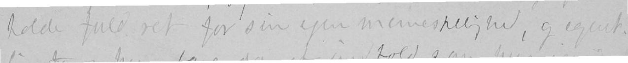holde fuld ret for sin egen menneskelighed, og egent-
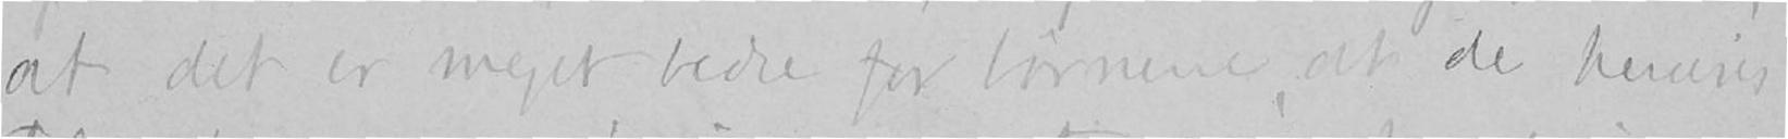at det er meget bedre for børnene, at de henvises
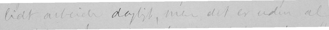lidt arbeide dagligt, men det er uden al
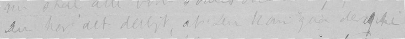Du har det deiligt, at Du kan gaa deroppe
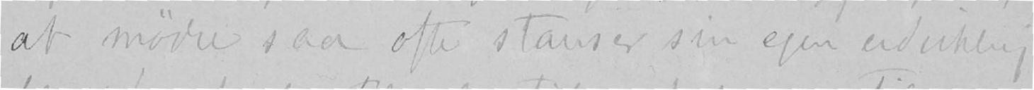at mødre saa ofte stanser sin egen udvikling
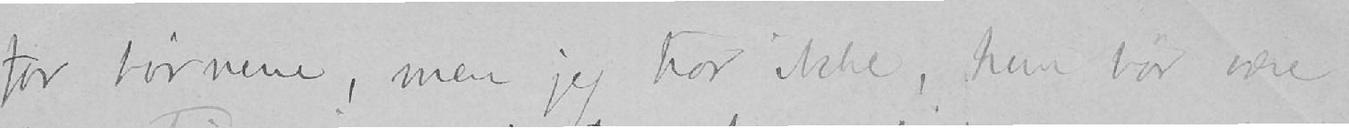for børnene, men jeg tror ikke, hun bør være
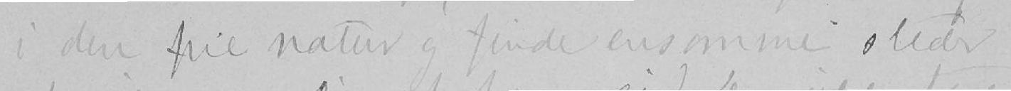i den frie natur og finde ensomme steder
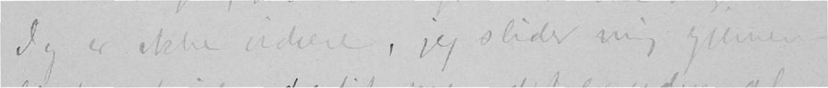Jeg er ikke videre, jeg slider mig gjennem
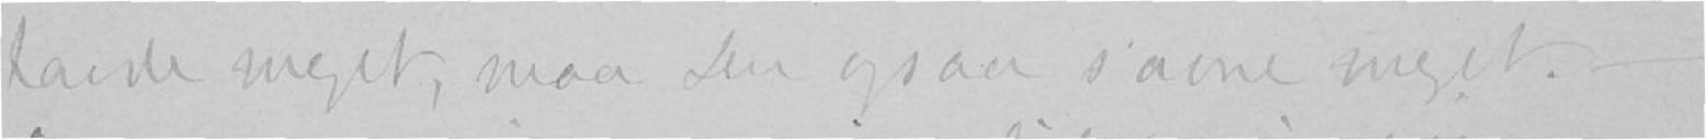havde meget, maa Du ogsaa savne meget. –
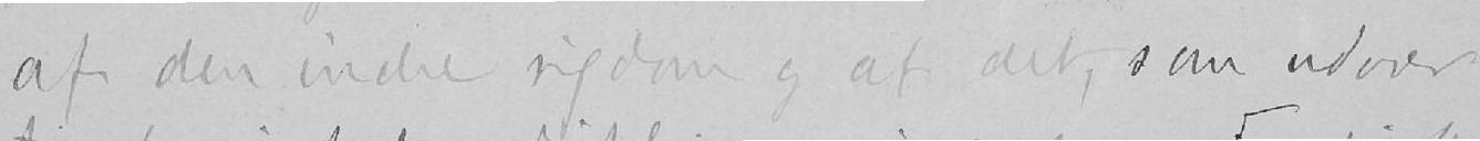af den indre rigdom og af det, som udover
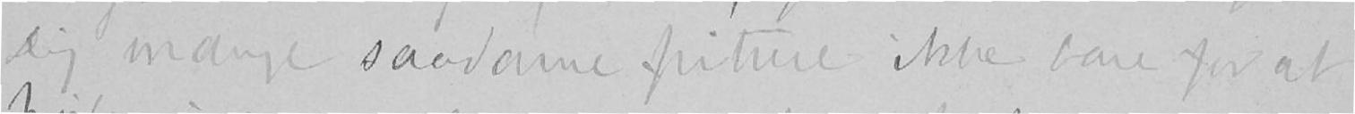Dig mange saadaane friture ikke bare for at
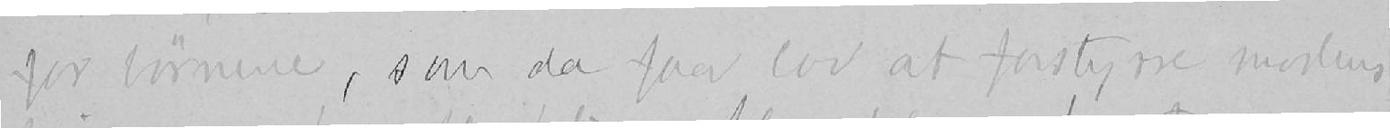for børnene, som da faa lov at forstyrre moders
